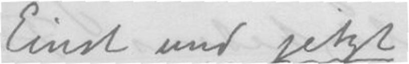Einst und jetzt
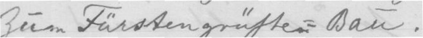Zum Färstengrüften=Bau.
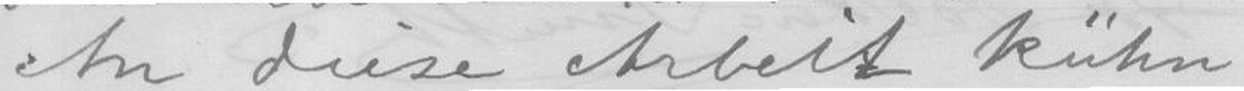An diese Arbeit kühn
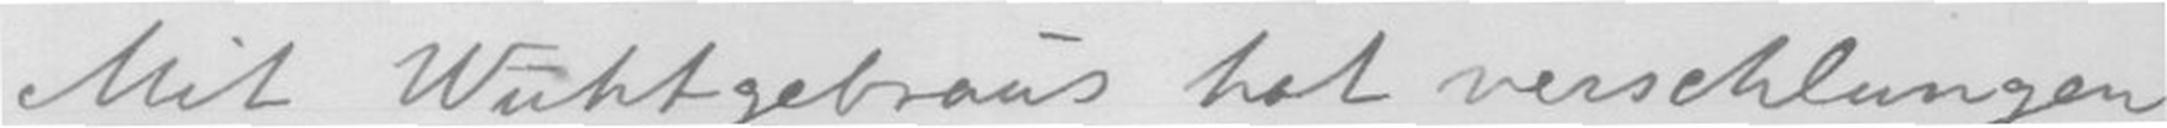Mit Wuhtgebraus hat verschlungen
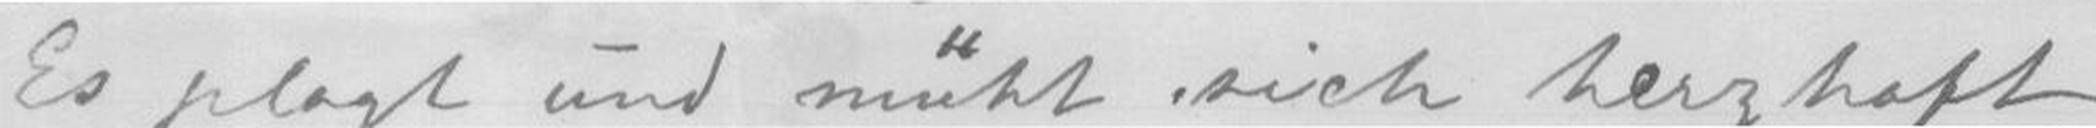Es plagt und müht sich herzhaft
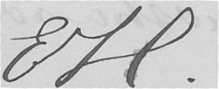EH.
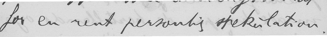for en rent personlig spekulation.
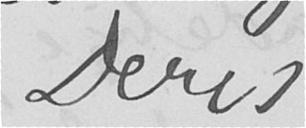Deres
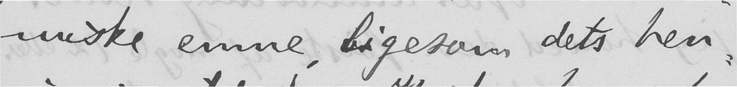miske emne, ligesom dets hen-
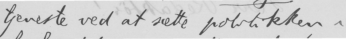tjeneste ved at sætte politikken i
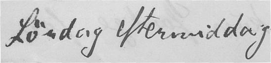Lørdag eftermiddag.
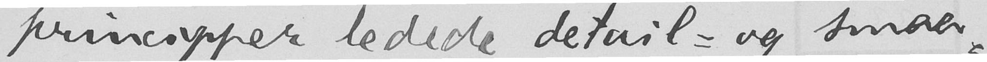principper ledede detail- og smaa-
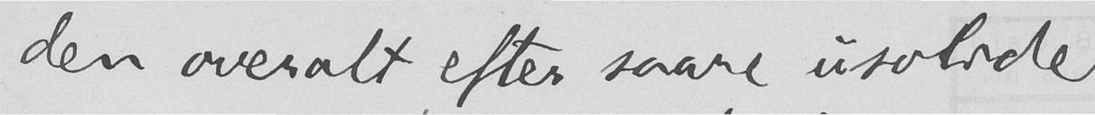den overalt efter saare usolide
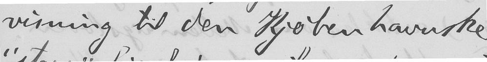visning til den Kjøbenhavnske
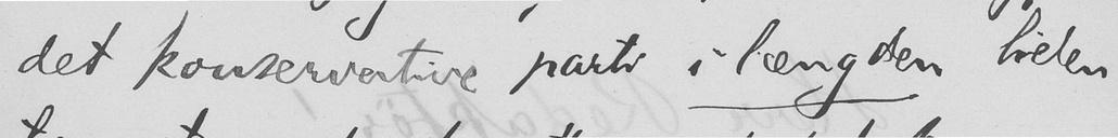det konservative parti i længden liden
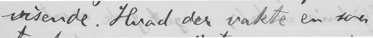visende. Hvad der vakte en saa
